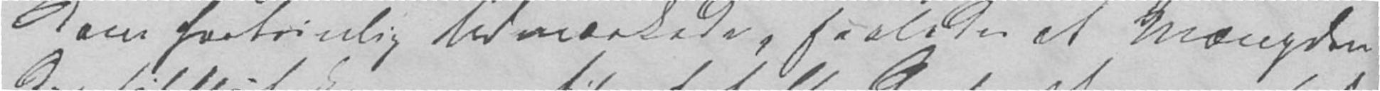dom fortrinlig Udmærkede, saaledes at Mængden
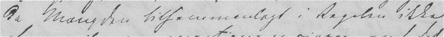da Mængden tilsammenlagt i Regelen ikke
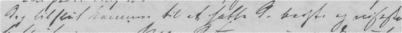dog tilslut kommer til at fatte de bedste og viseste
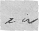en
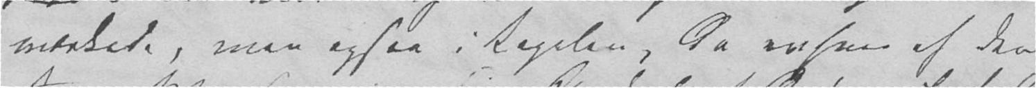mærkede, men ogsaa i Regelen, da enhver af den
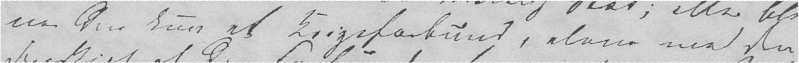ver den kun et Krigsforbund, alene med den
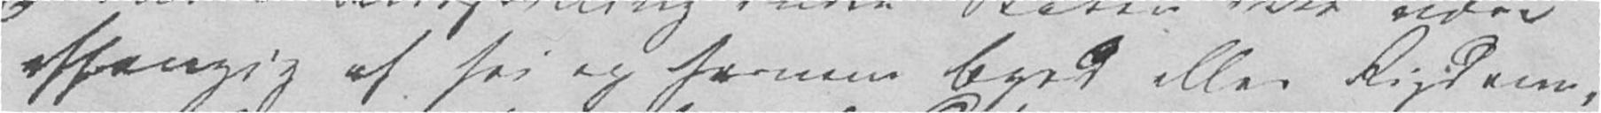afhængig af høi og fornem Byrd eller Rigdom,
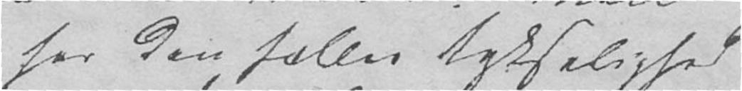har den fælles Lyksalighed
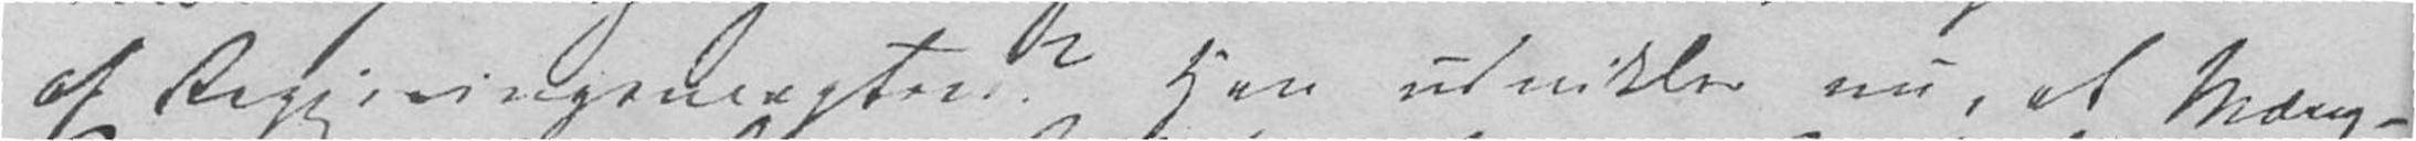at Regjeringsmagten? Han udvikler nu, at Mæng-
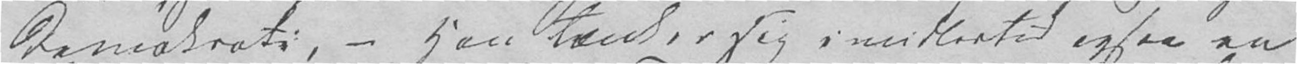Demokrati, – han tænker sig imidlertid ogsaa en
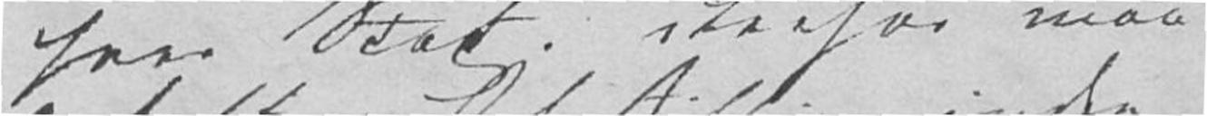hver Stat. Derfor maa
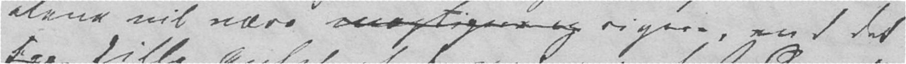alene vil være mægtigere og rigere, end det
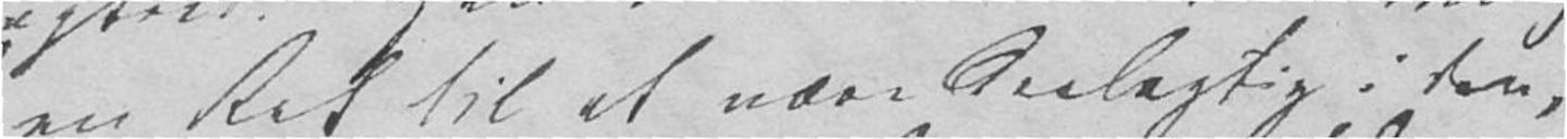en Ret til at være deelagtig i den,
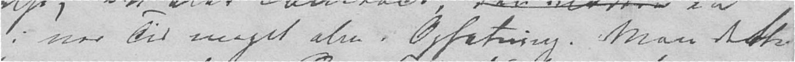i vor Tid meget alm. Opfatning. Men dette
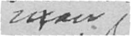man
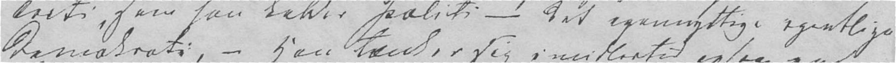crati, som han kalder Politi – det egenyttige egentlige
